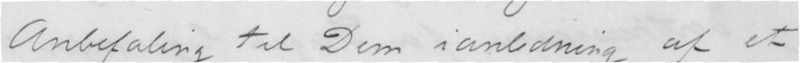Anbefaling til Dem ianledning af et
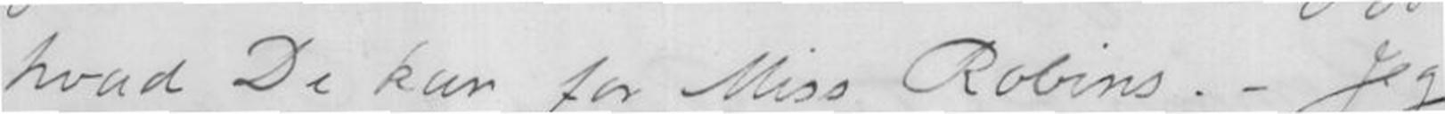hvad De kan for Miss Robins. - Jeg
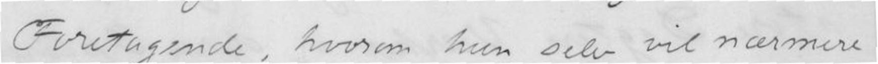Foretagende, hvorsom hun selv vil nærmere
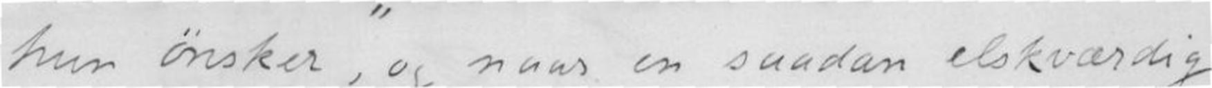hun ønsker," og naar en saadan elskværdig
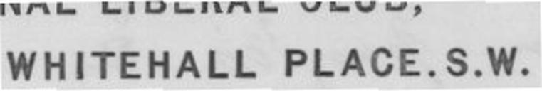WHITEHALL PLACE.S.W.
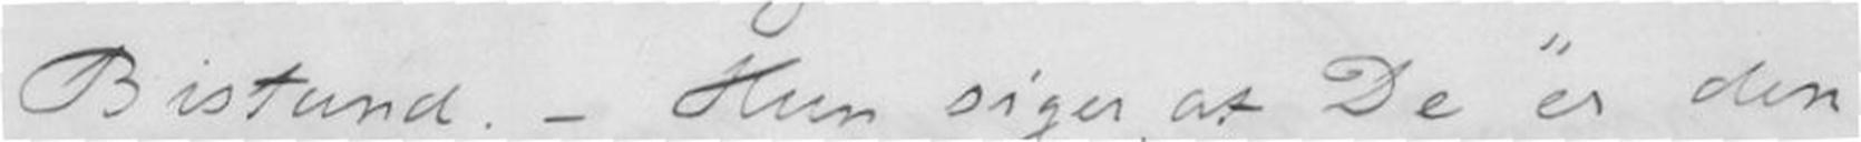Bistand. - Hun siger, at De "er den
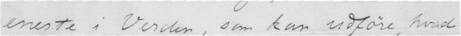eneste i Verden, som kan udføre, hvad
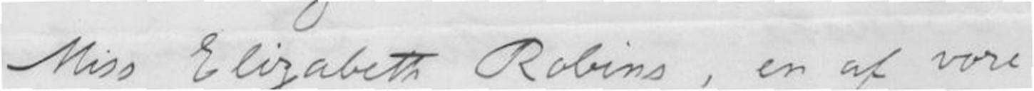Miss Elzabeth Robins, en af vore
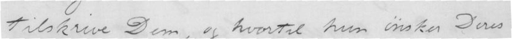tilskrive Dem, og hvortil hun ønsker Deres
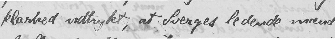klarhed udtrykt, at Sverges ledende mænd
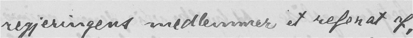regjeringens medlemmer et referat af,
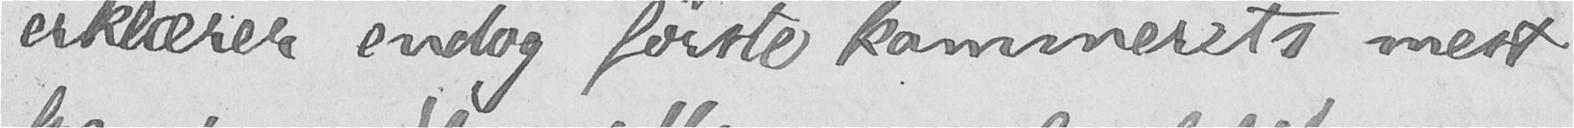erklærer endog første kammerets mest
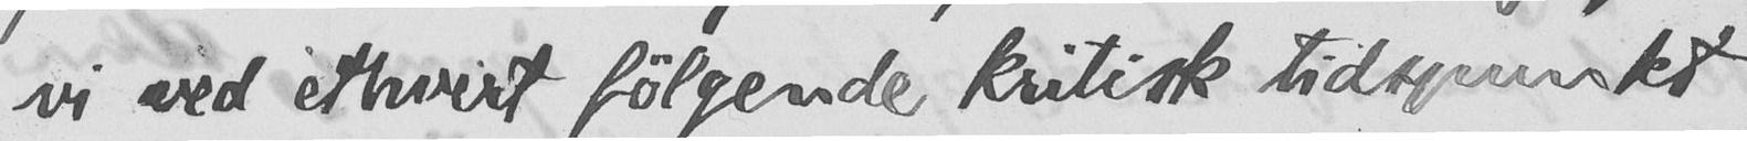vi ved ethvert følgende kritisk tidspunkt
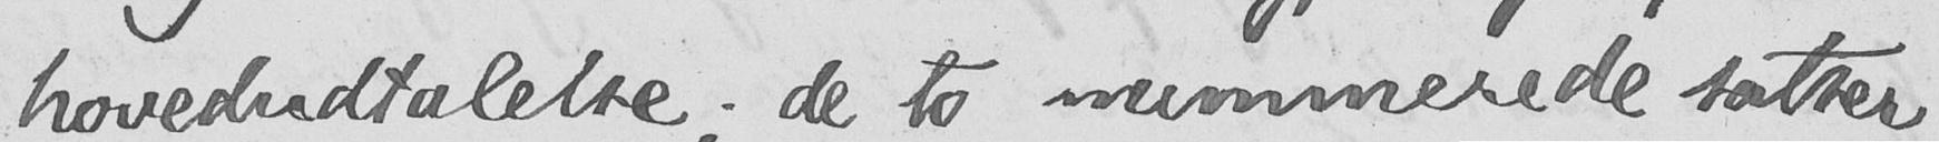hovedudtalelse; de to nummerede satser
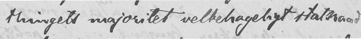thingets majoritet velbehageligt statsraad
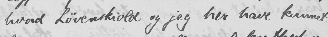hvad Løvenskiold og jeg her have kunnet
